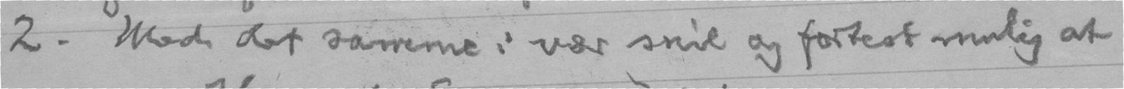2. Med det samme: vær snil og fortest mulig at
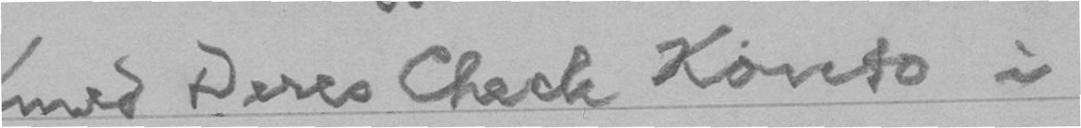med Deres Check Konto i
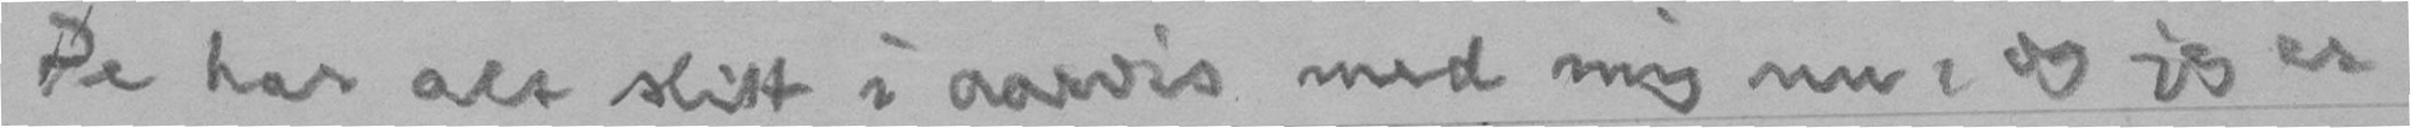De har alt slitt i aarvis med mig nu, og jeg er
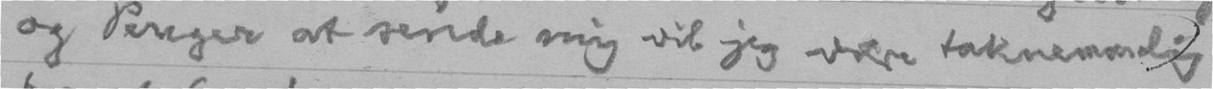og Penger at sende mig vil jeg være taknemmelig
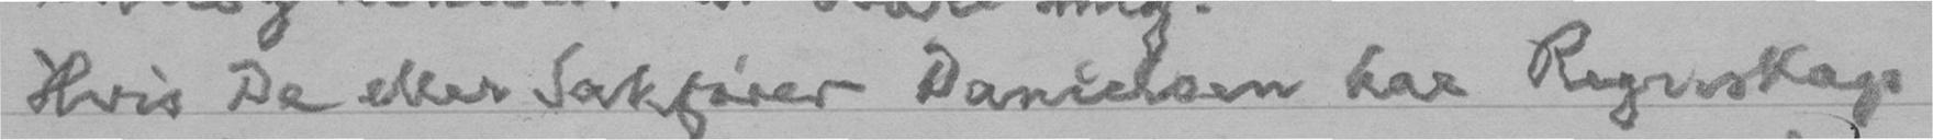Hvis De eller Sakfører Danielsen har Regnskap
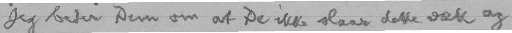Jeg beder Dem om at De ikke slaar dette væk og
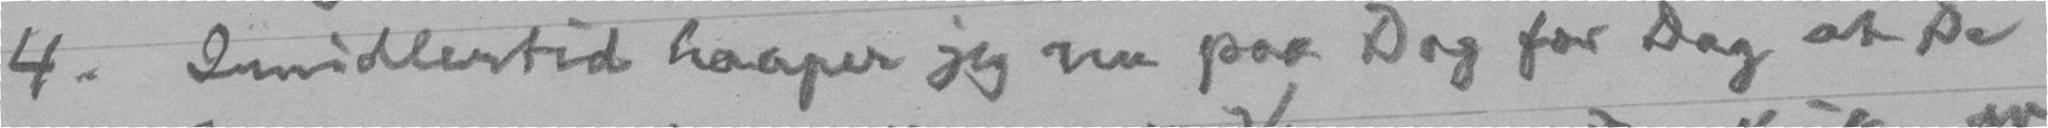4. Imidlertid haaper jeg nu paa Dag for Dag at De
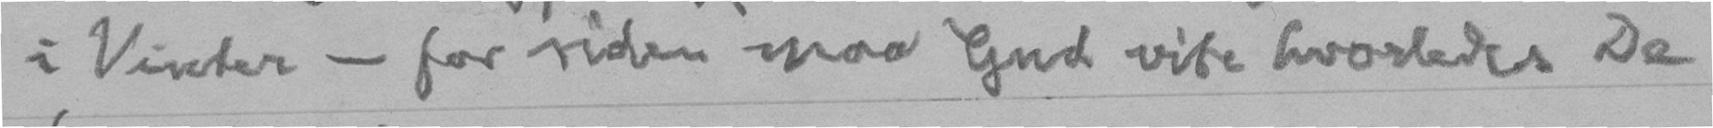i Vinter - for siden maa Gud vite hvorledes De
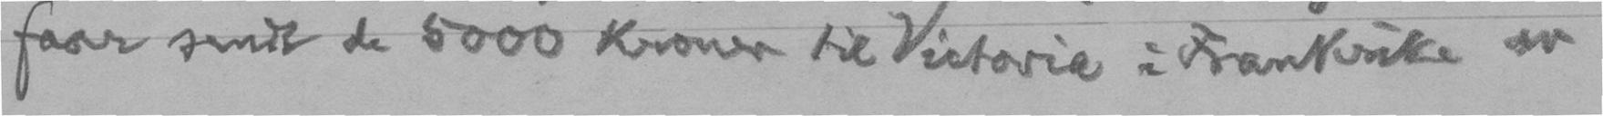faar sendt de 5000 Kroner til Victoria i Frankrike av
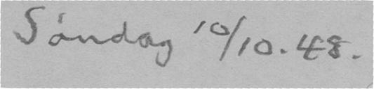Søndag 10/10. 48.
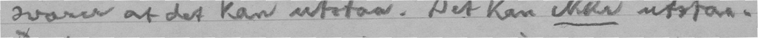svarer at det kan utstaa. Det kan ikke utstaa.
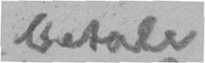betale
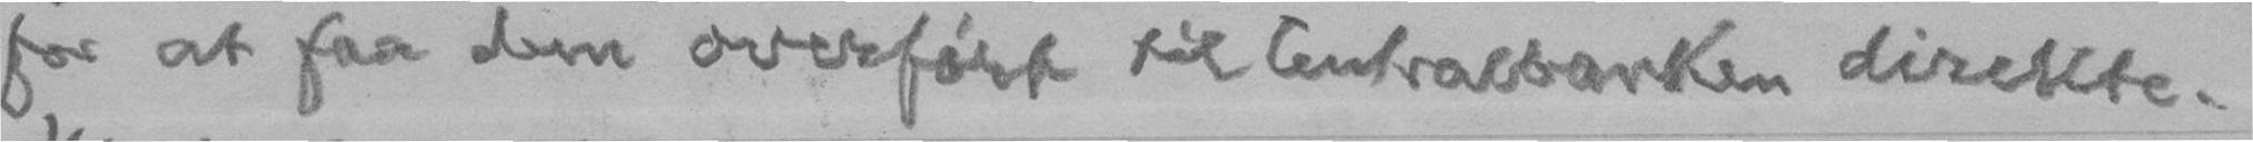for at faa dem overført til Centralbanken direkte.
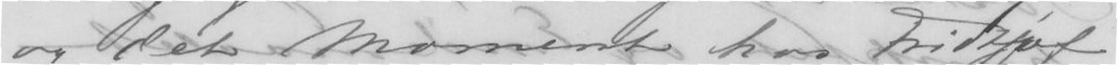og det Moment hos Fridtjof
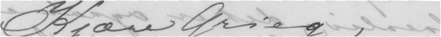Kjære Grieg,
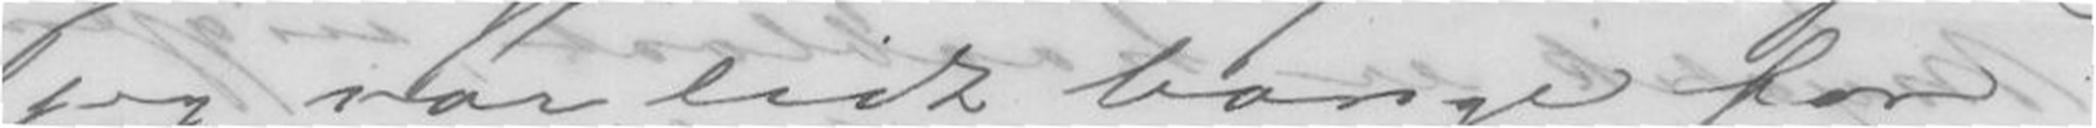jeg var lidt bange for
